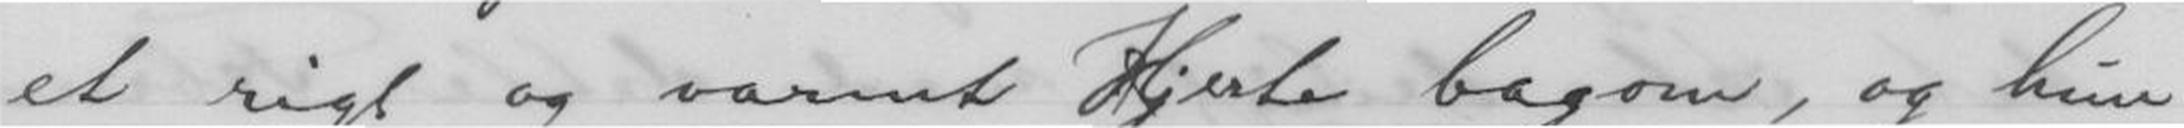et rigt og varmt Hjerte bagom, og hun
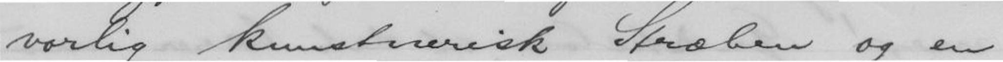vorlig kunstnerisk Stræben og en
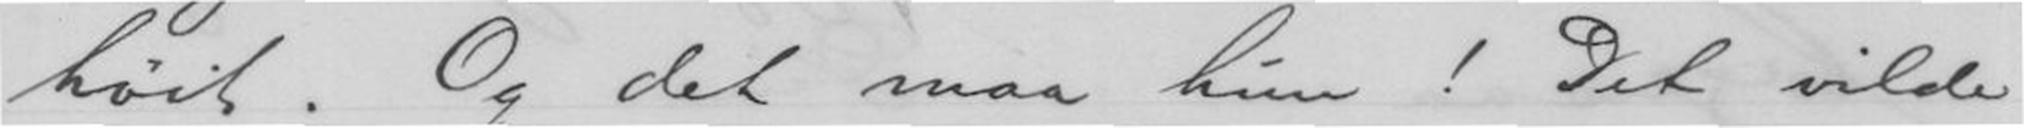høit. Og det maa hun! Det vilde
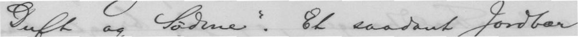Duft og Sødne". Et saadant Jordbær
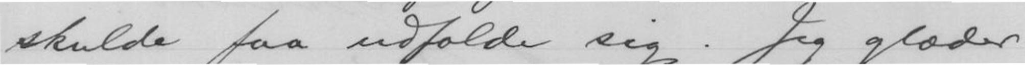skulde faa udfolde sig. Jeg glæder
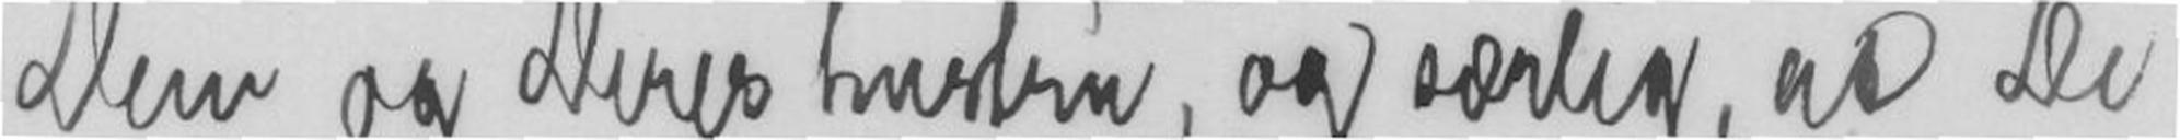Dem og Deres hustru, og særlig, at De
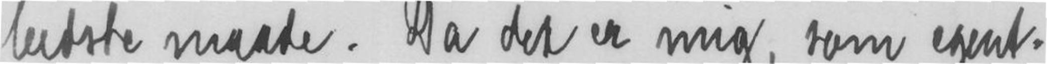bedste maade. Da det er mig, som egen-
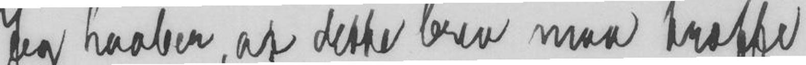Jeg haaber, at dette brev maa træffe
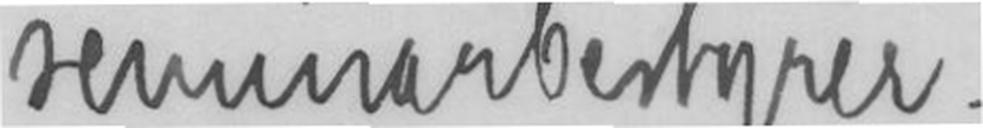seminarbestyrer.
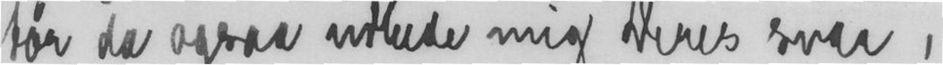tør da ogsaa udbede mig Deres svar,
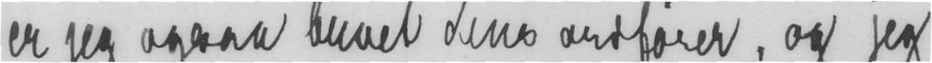er jeg ogsaa blevet dens ordfører, og jeg
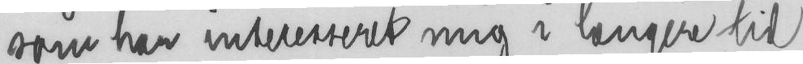som har interesseret mig i længre tid,
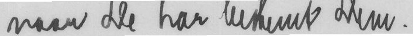naar De har bestemt Dem.
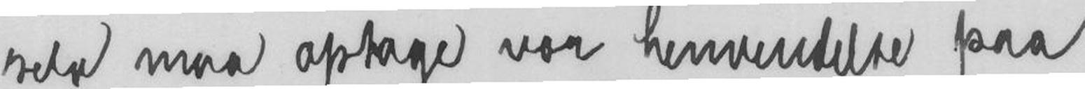selv maa optage vor henvendelse paa
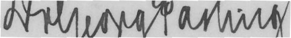Dr. Georg Fasting
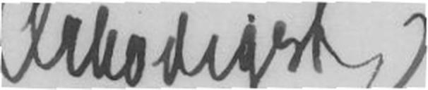Ærbødigst
